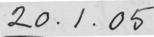20.1.05
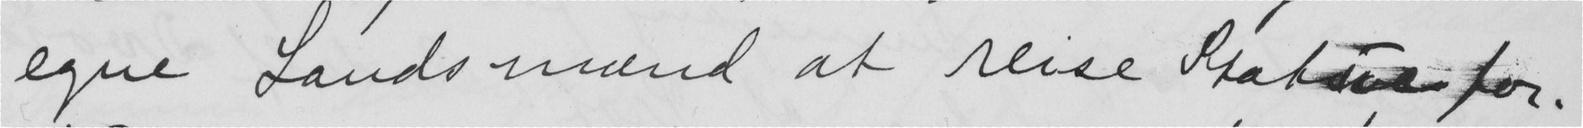egne Landsmænd at reise Statue for.
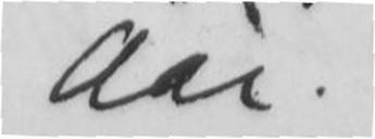Aar.
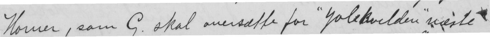Homer, som G. skal oversætte for Jolekvelden næste
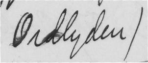Ordlyden)
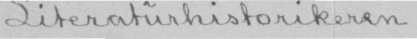Literaturhistorikeren
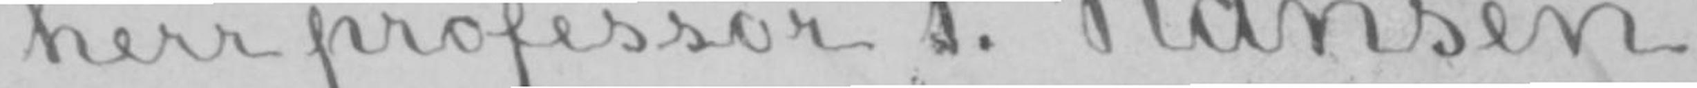herr professor P. Hansen.
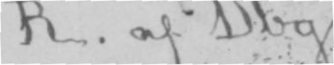R. af Dbg.
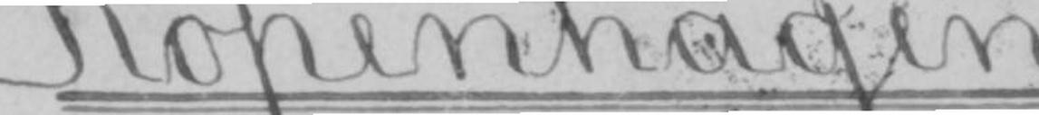Kopenhagen.
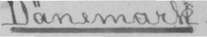(Dänemark.)
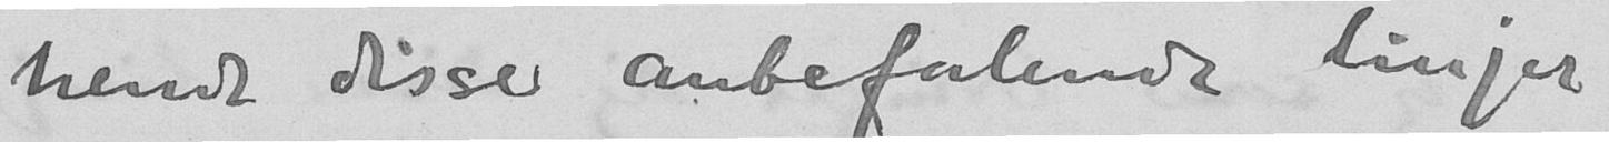hende disse anbefalende linjer
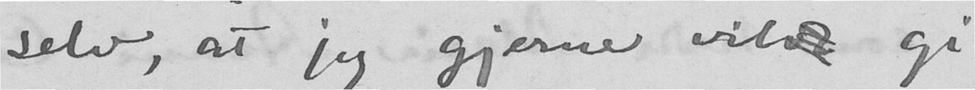selv, at jeg gjerne vilde gi
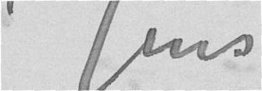Jens
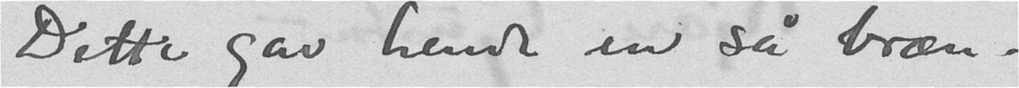Dette gav hende en så bræn-
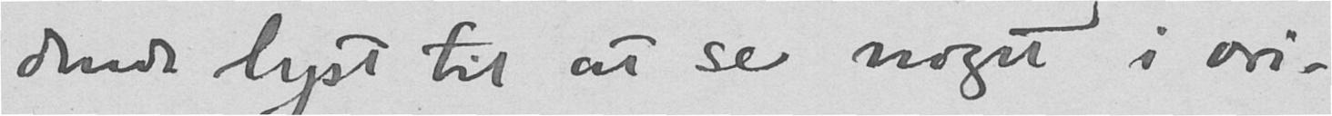dende lyst til at se noget i ori-
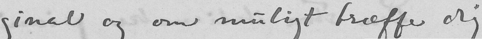ginal og om muligt træffe dig
